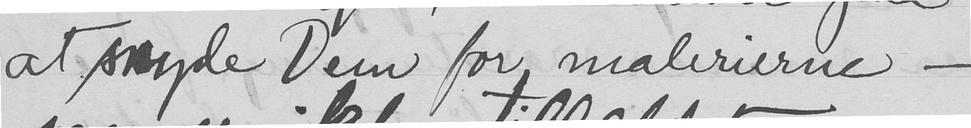at snyde Dem for malerierne -
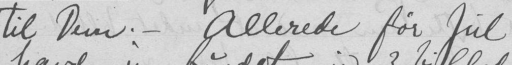til Dem. - Allerede før jul
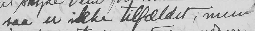saa er ikke tilfældet; men
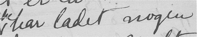har ladet nogen
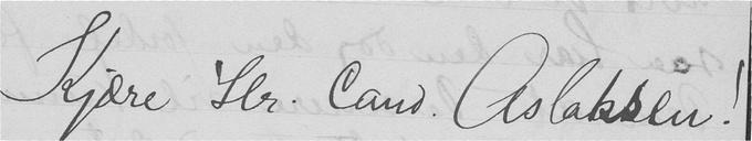Kjære Hr. Cand. Aslaksen!
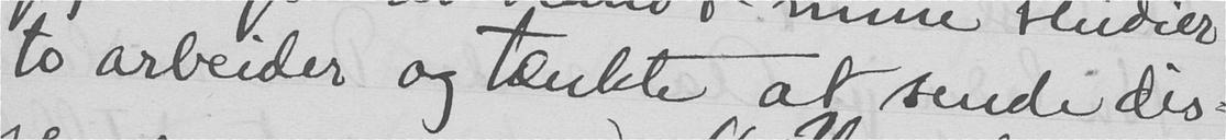to arbeider og tænkte at sende dis-
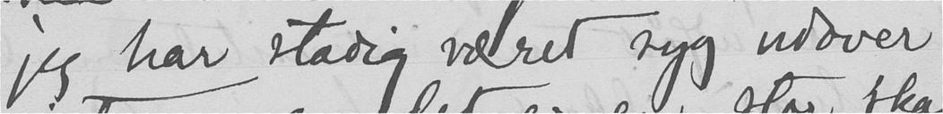jeg har stadig været syg udover
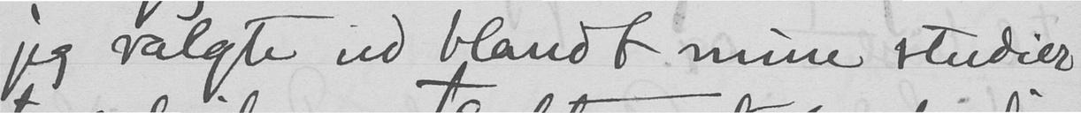jeg valgte ud blandt mine studier
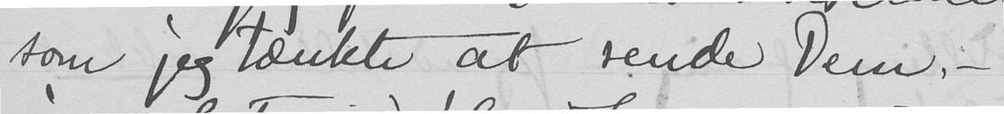som jeg tænkte at sende Dem, -
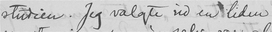studien. Jeg valgte ud en liden
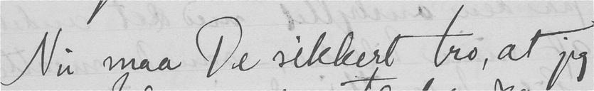Nu maa De sikkert tro, at jeg
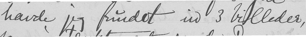havde jeg fundet ud 3 billeder,
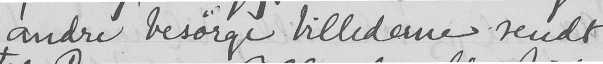andre besørge billederne sendt
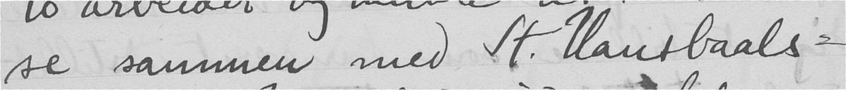se sammen med St. Hansbaals-
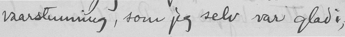vaarstemning, som jeg selv var glad i;
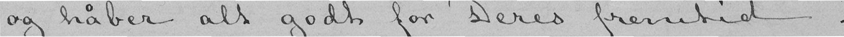og håber alt godt for Deres fremtid.
